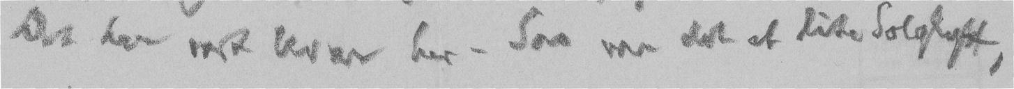Det har vært Uvær her. Saa var det et lite Solglytt,
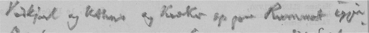Vedskjul og Uthus og kræker op paa Rummet igjen.
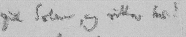gik Solen, og sitter her!
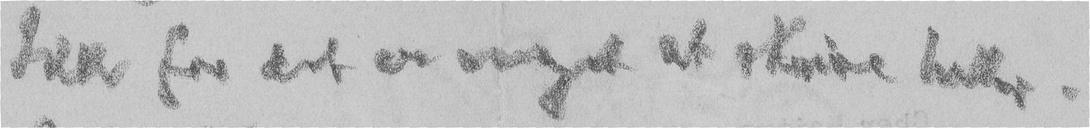Ikke for det er noget at skrive heller.
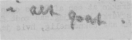i alt godt.
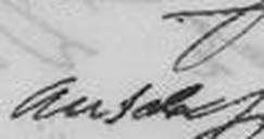Antal
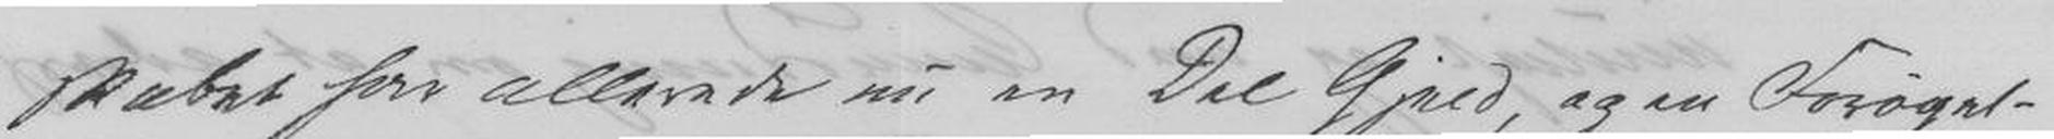skabet have allerede nu en Del Gjeld, og en Forøgel-
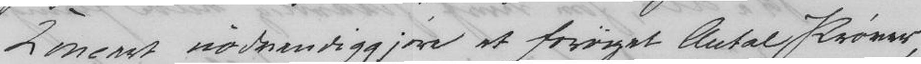Koncert nødvendiggjøre et forøget Antal Prøver,
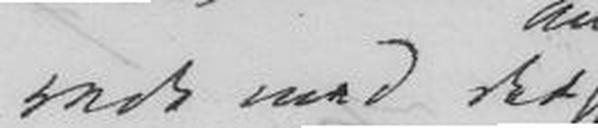rede med det
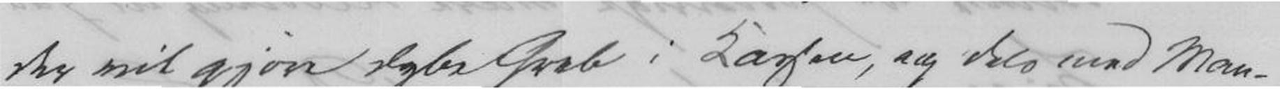der vil gjøre dybe Greb i Kassen, og dels med Man-
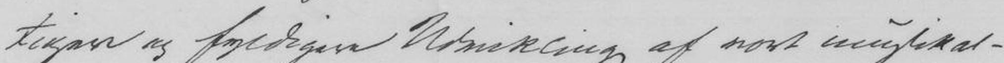tigere og fyldigere Udvikling af vort musikal-
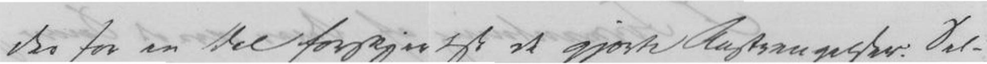der for en Del forskedste de gjorde Anstrengelser. Sel-
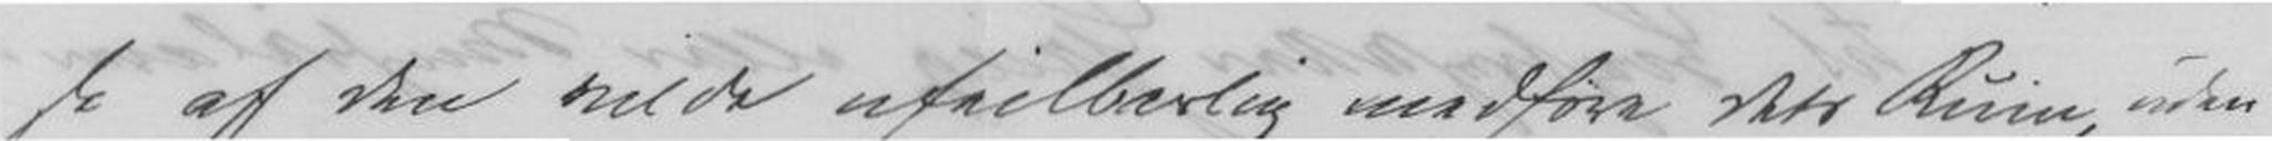se af den vilde ufeilbarlig medføre dets Ruin, uden
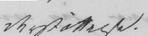derstøttelse.
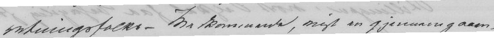retningsfolks- Indkommende, vist en gjennemgaaen-
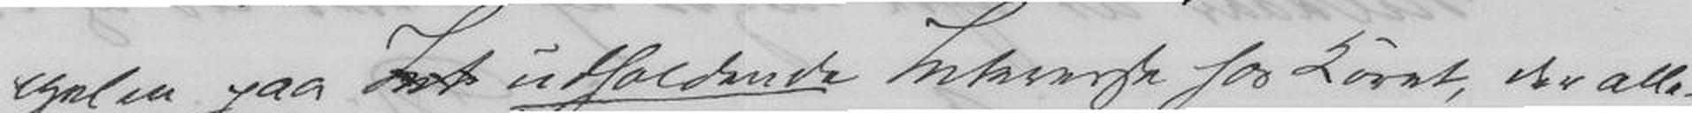gelen paa Int utholdende Interesse for Koret, der alle-
